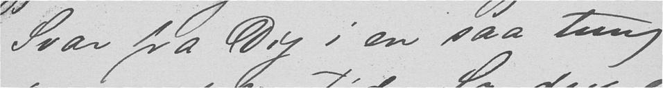Svar fra Dig i en saa tung
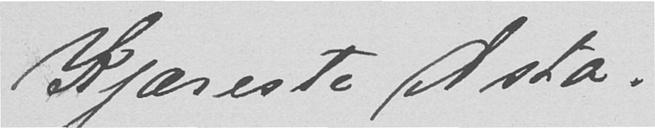Kjæreste Asta.
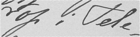op i Tele-
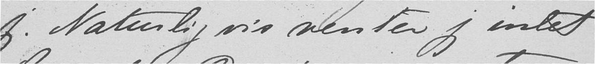jeg. Naturligvis venter jeg intet
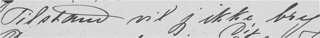Tilstand vil jeg ikke bry
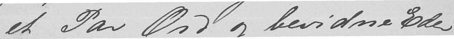et Par Ord og bevidne Eder
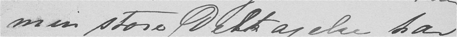min store Deltagelse har
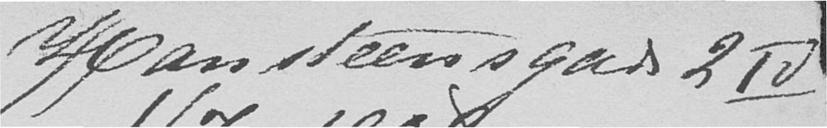Hansteensgade 2 IV
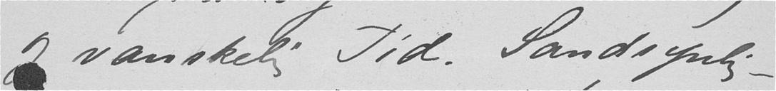og vanskelig Tid. Sandsynlig-
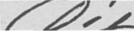Dig
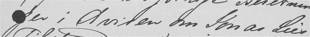ger i Avisen om Jonas Lies
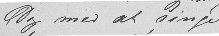Dig med at ringe
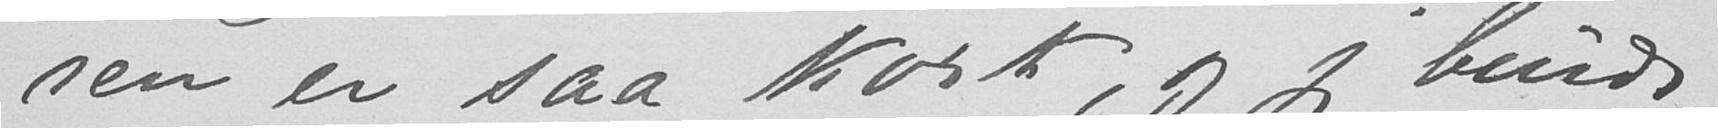ren er saa kort, og jeg kunde
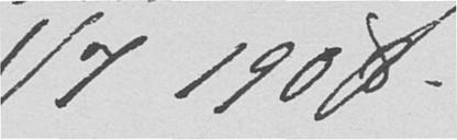1/7 1908.
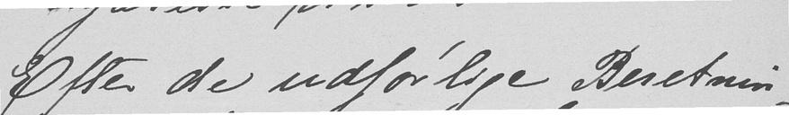Efter de udførlige Beretnin-
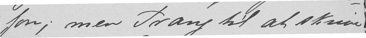fon; men Trang til at skrive
